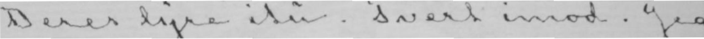Deres lyre itu. Tvertimod. Jeg
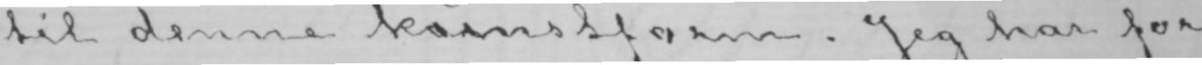til denne kunstform. Jeg har for
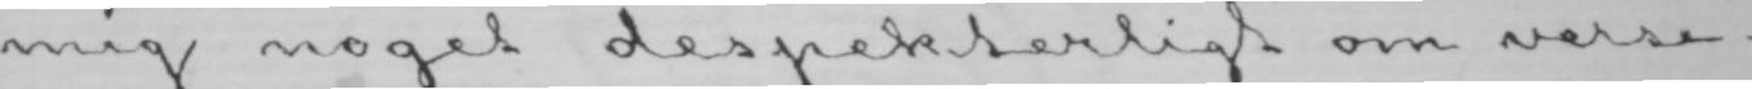mig noget despekterligt om verse-
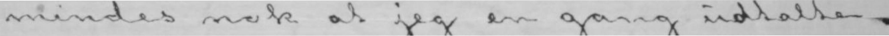mindes nok, at jeg en gang udtalte
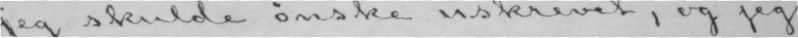jeg skulde ønske uskrevet, og jeg
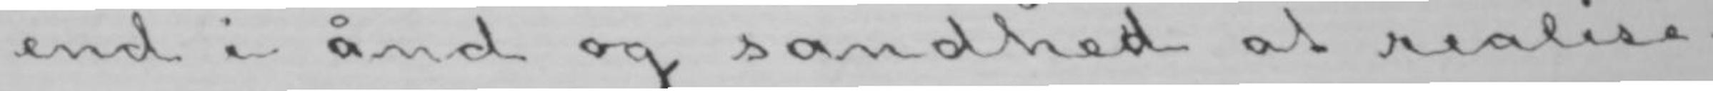end i ånd og sandhed at realise-
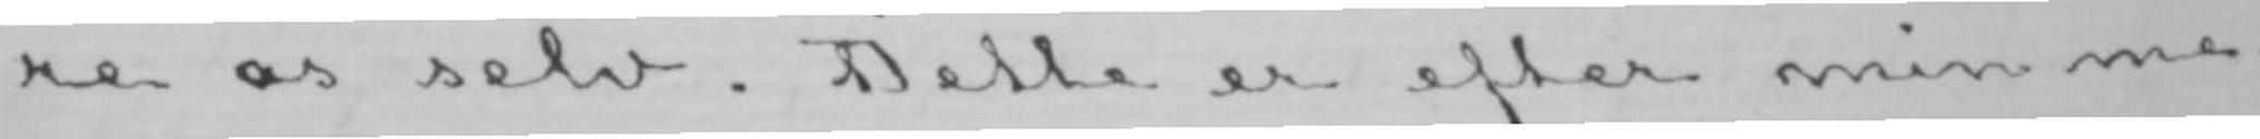re os selv. Dette er efter min me-
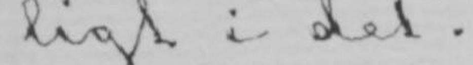ligt i det.
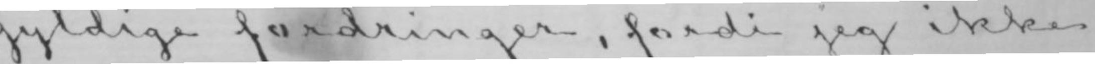gyldige fordringer, fordi jeg ikke
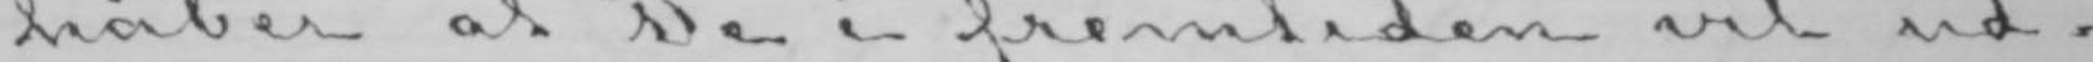håber at De i fremtiden vil ud-
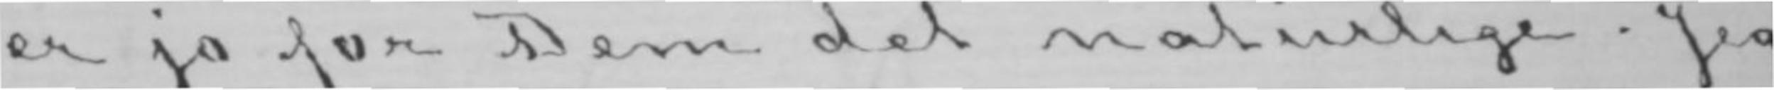er jo for Dem det naturlige. Jeg
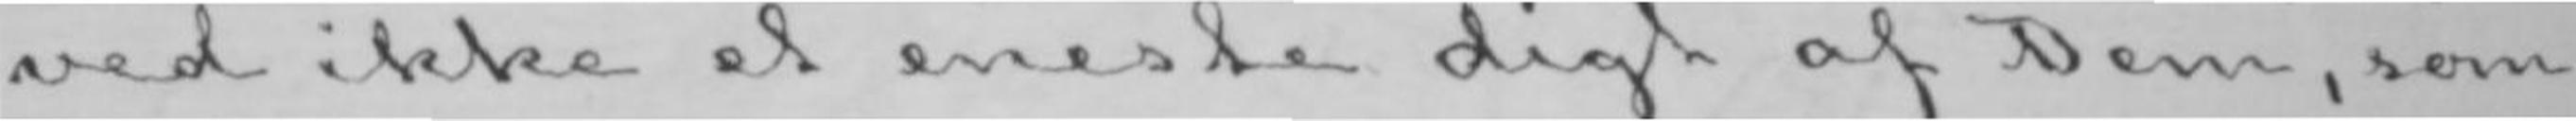ved ikke et eneste digt af Dem, som
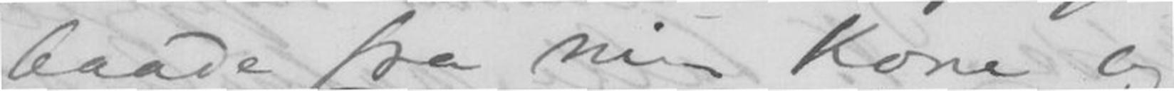og
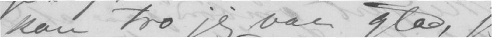kan tro jeg var glad, jeg
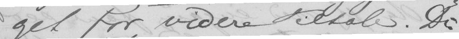get for videre Tiltale. Du
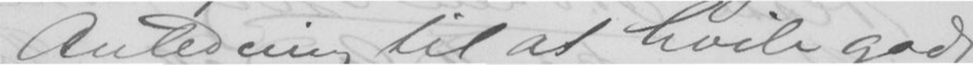Anledning til at hvile godt
